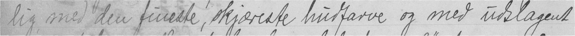lig, med den fineste, skjæreste hudfarve og med udslagent
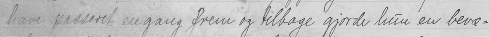have passeret en gang frem og tilbage gjorde hun en bevæ-
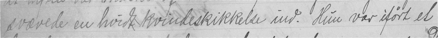svævede en hvidt kvindeskikkelse ind. Hun var iført et
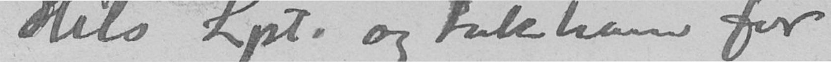Hils Lpt. og tak ham for
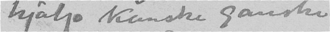hjalp kanske ganske
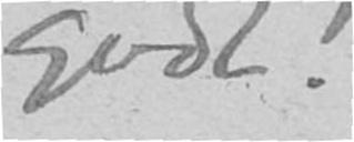godt!
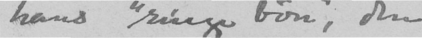hans "ringe bøn", den
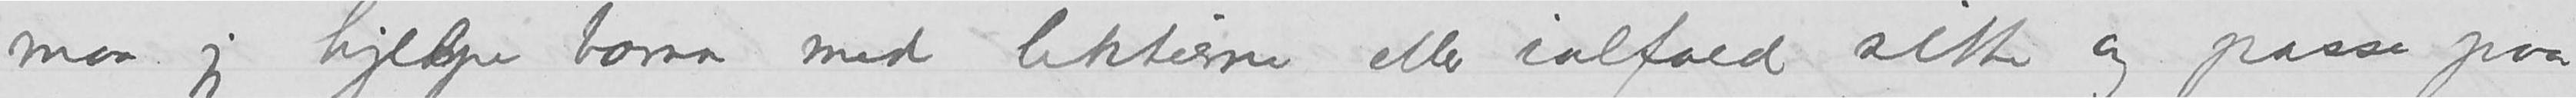maa jeg hjelpe barna med lektierne eller ialfald sitte og passe paa
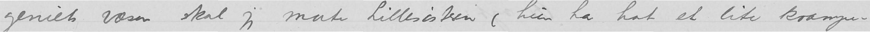geniets væsen skal jeg mate Lillesøsteren (hun har hat et lite krampe-
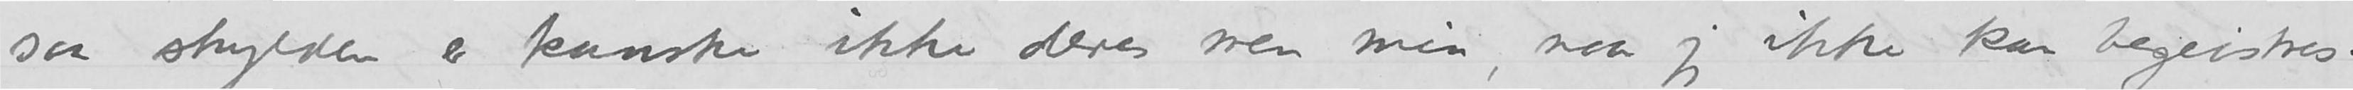saa skylden er kanske ikke deres men min, naar jeg ikke kan begeistres.
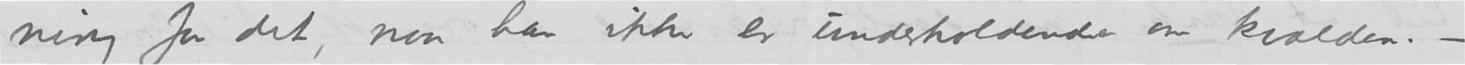ning for det, naar han ikke er underholdende om kvælden. –
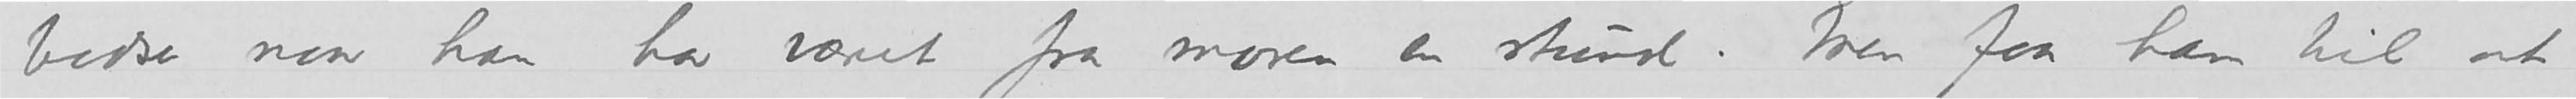bedre naar han har været fra moren en stund. Men faa ham til at
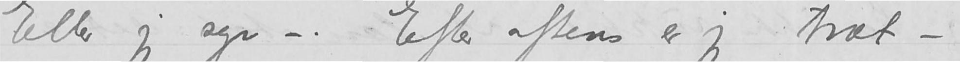Eller jeg sygr –. Efter aftens er jeg træt –.
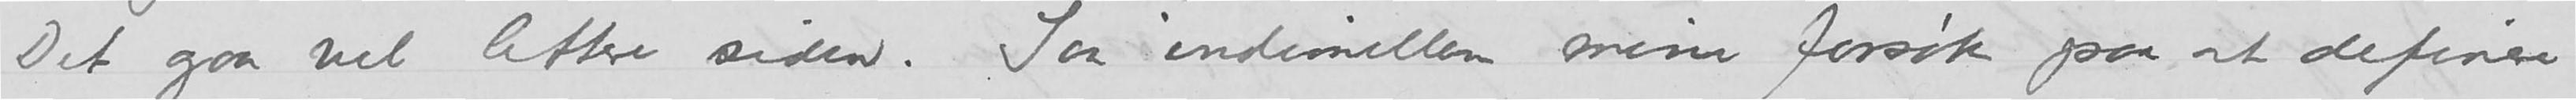Det gaar vel lettere siden. Saa indimellem mine forsøk paa at definere
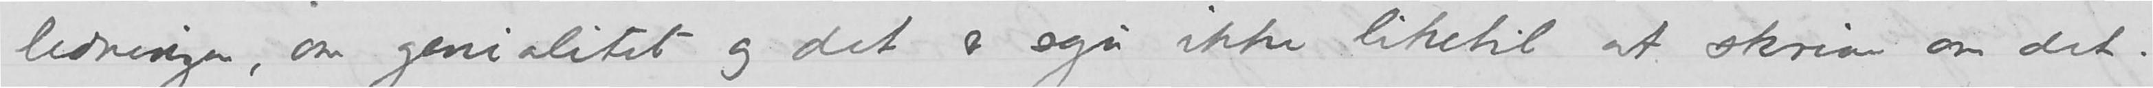ledningen, om genialitet og det er sgu ikke liketil at skrive om det.
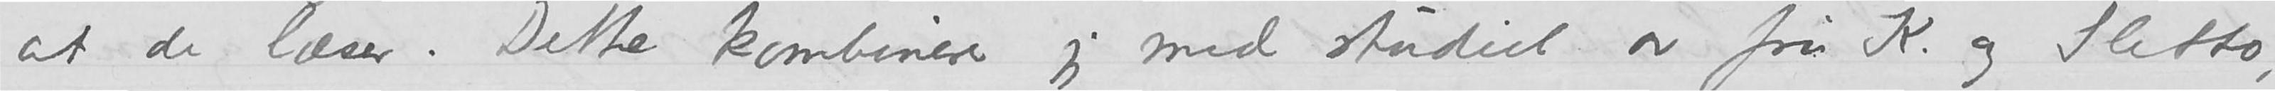at de læser. Dette kombinerer jeg med studiet av fru K. og Sletto,
